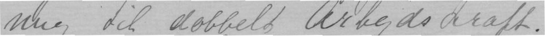mig til dobbelt Arbeidskraft.
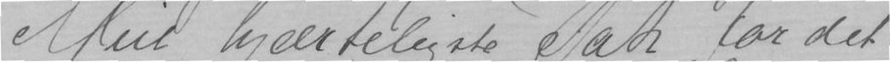Mit hjærteligste Tak for det
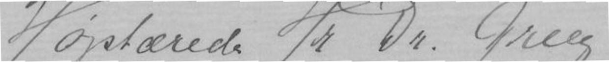Højstærede Hr. Dr. Grieg
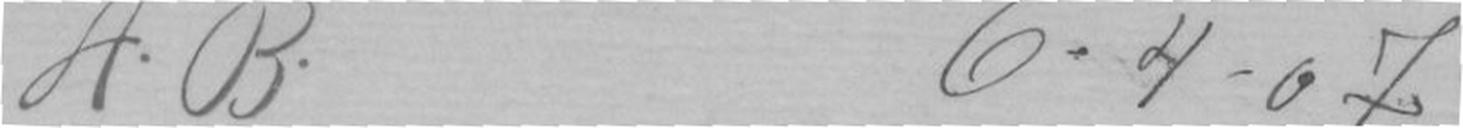A.B.6.4.07
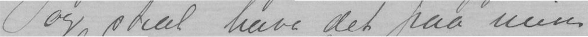og skal have det paa min
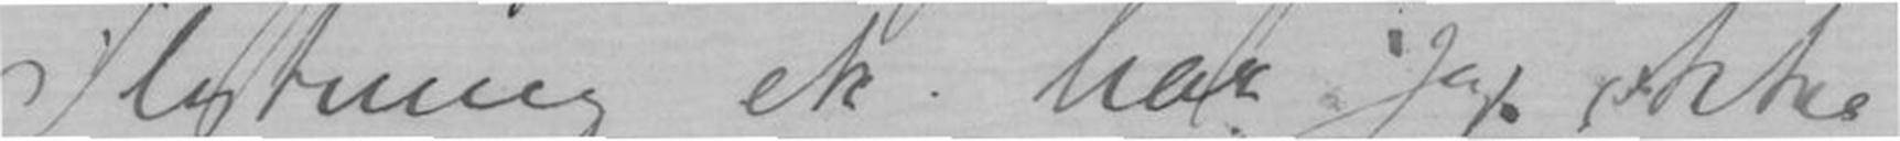Flytning ek. har jeg ikke
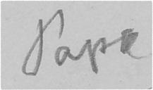Papa
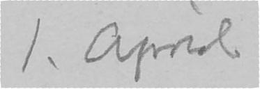1. April
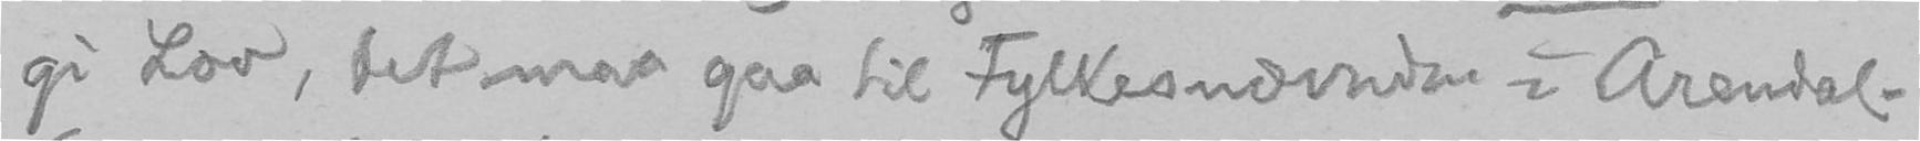gi Lov, det maa gaa til Fylkesnæmden i Arendal.
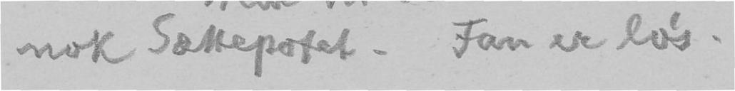nok Sættepotet. Fan er løs.
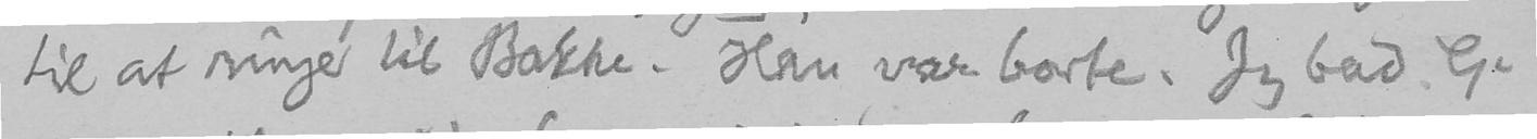til at ringe til Bakke. Han var borte. Jeg bad G.
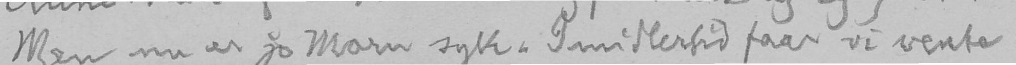Men nu er jo Morn syk. Imidlertid faar vi vente
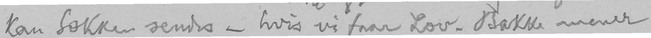kan Sækken sendes - hvis vi faar Lov. Bakke mener
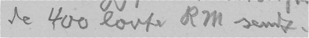de 400 lovte RM sendt.
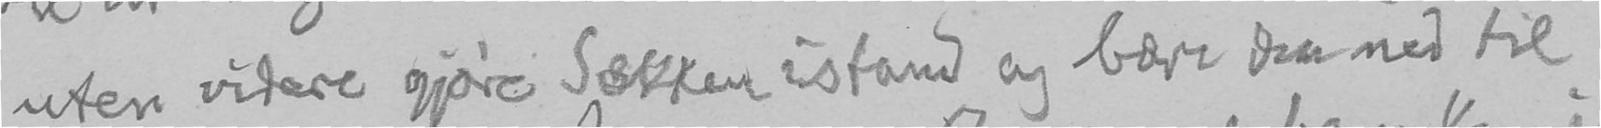uten videre gjøre Sækken istand og bære den ned til
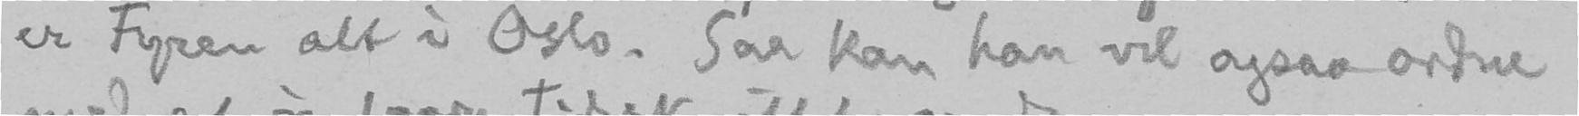er Fyren alt i Oslo. Saa kan han vel ogsaa ordne
